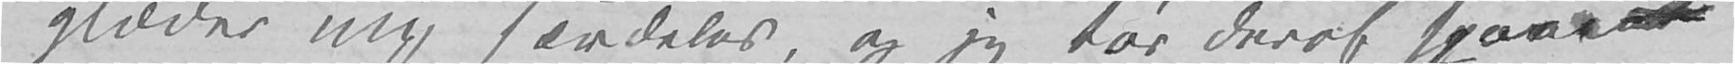glæder mig særdeles, og jeg tør deraf spaae at
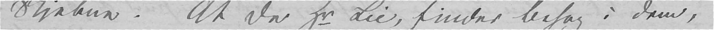Skjebne. At De Hr Lie, finder Behag i dem,
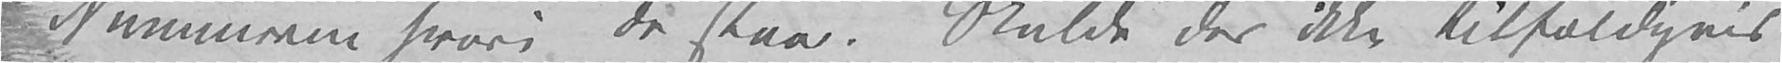Nummrene hvori de staa. Skulde der ikke tilfældigvis
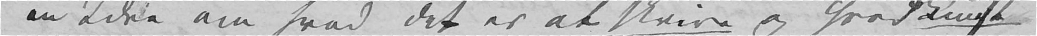en Idee om hvad det er at skrive og hvad Kunst
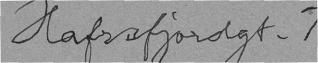Hafrsfjordgt. 7
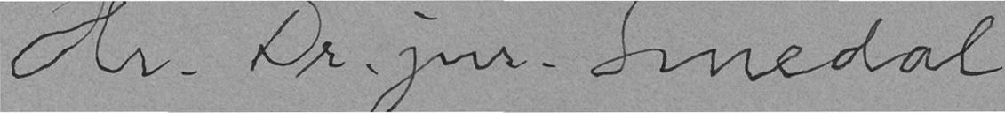Hr. Dr. jur. Smedal
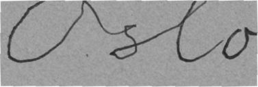Oslo
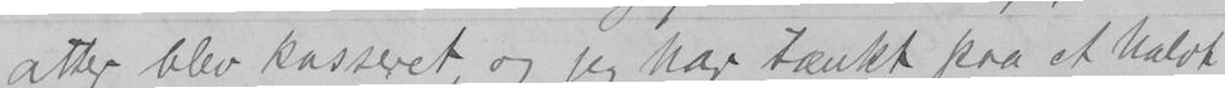atter blev kasseret, og jeg har tænkt paa et halvt
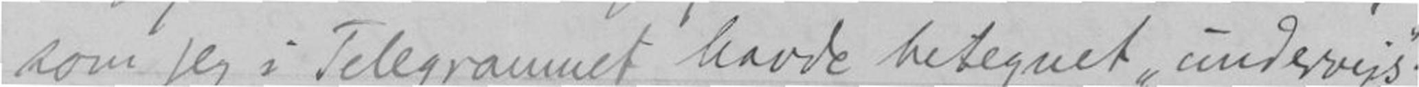som jeg i Telegrammet havde betegnet undervejs.
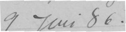9 Juni 86.
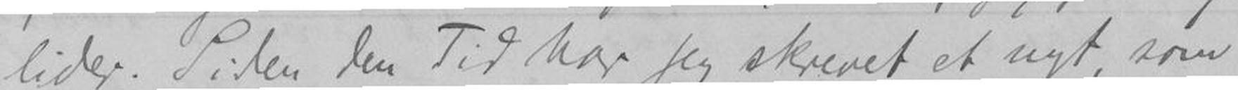lider. Siden den Tid har jeg skrevet et nyt, som
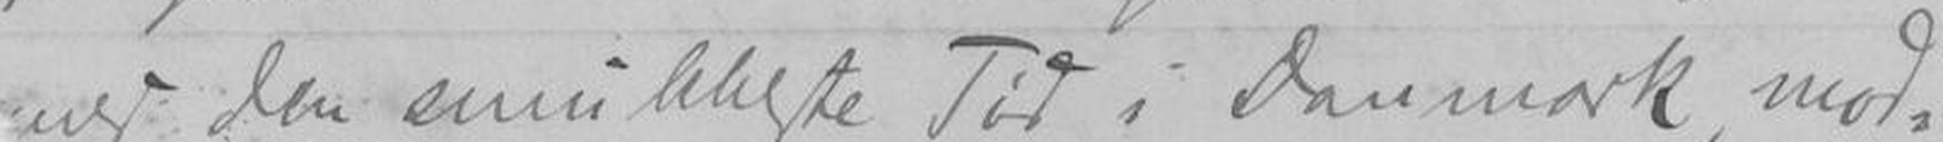den smukkligte Tid i Danmark, mod-
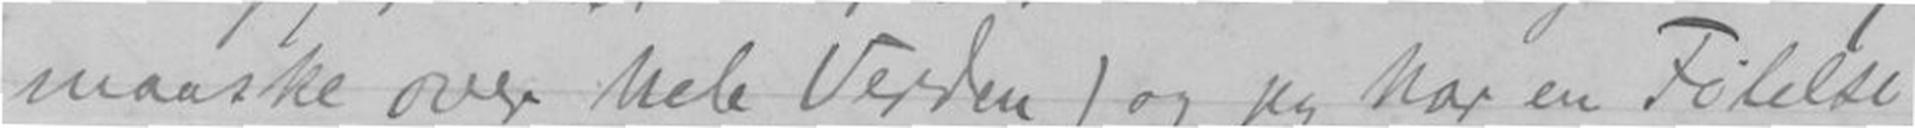maaske over hele Verden) og jeg har en Følelse
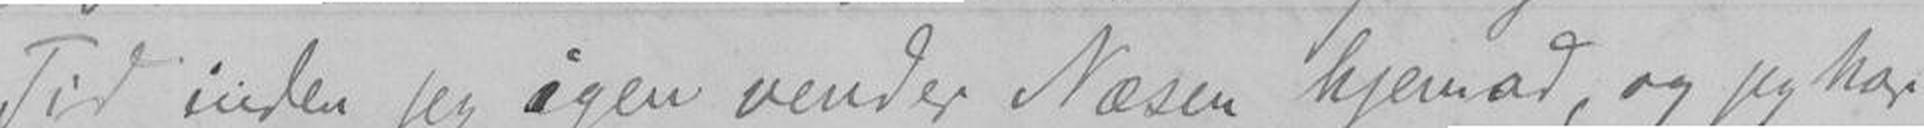Tid inden jeg igen vender Næsen hjemad, og jeg har
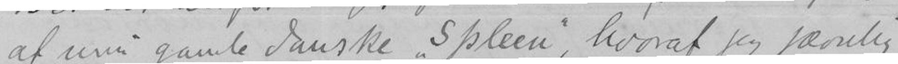af min gamle Danske Spleen, hvoraf jeg jævnlig
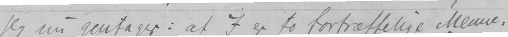jeg nu gentager: at I er to fortræffelige Menne-
